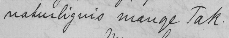naturligvis mange Tak.
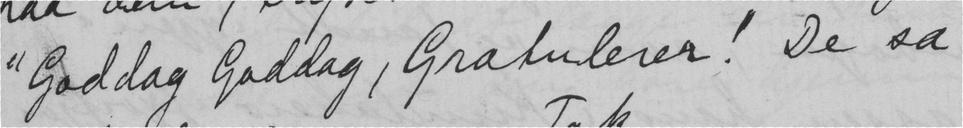"Goddag Goddag, Gratulerer! De sa
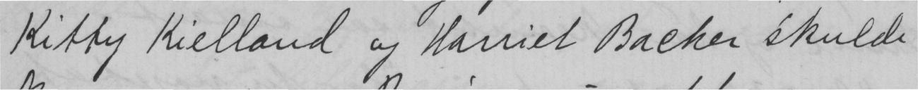Kitty Kielland og Harriet Backer skulde
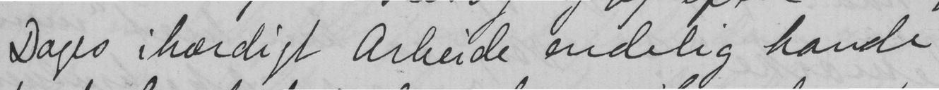Dages ihærdigt arbeide endelig havde
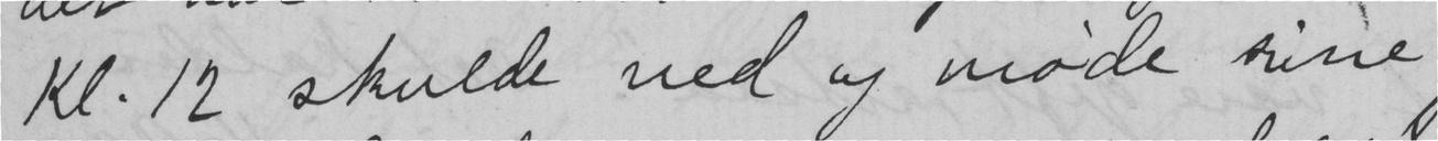Kl. 12 skulde ned og møde sine
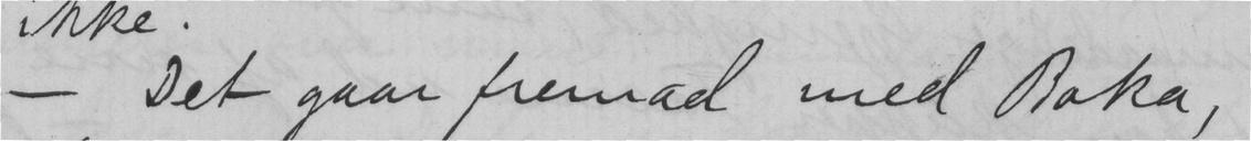- Det gaar fremad med Boka,
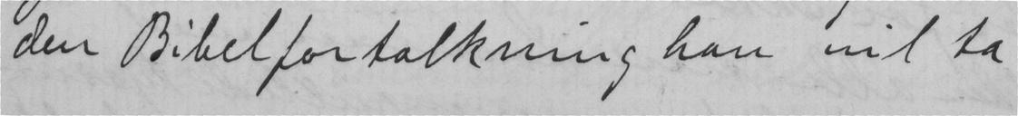den Bibelfortolkning han vil ta
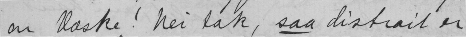en Væske! Nei tak, saa distrait er
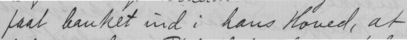faat banket ind i hans Hoved, at
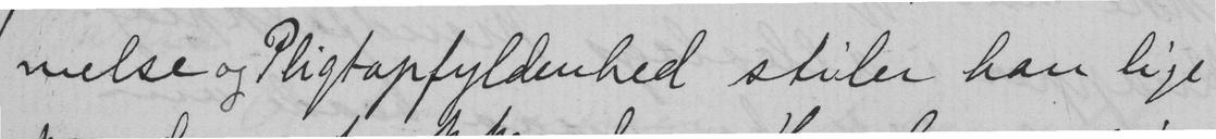melse og Pligtopfyldenhed stiler han lige
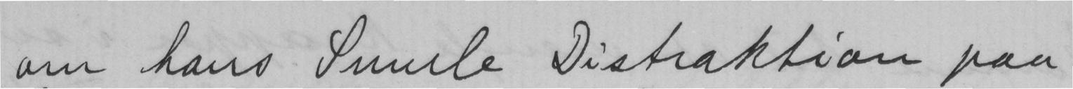om hans Smule Distraktion paa
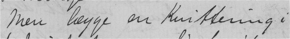Men lægge en Kvittering i
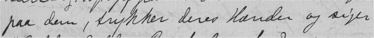paa dem, trykker deres Hænder og siger
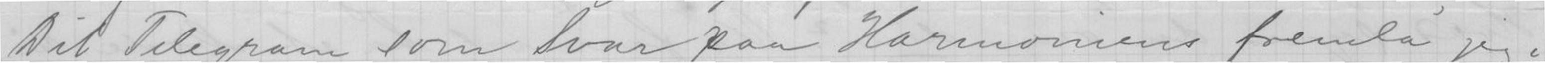Dit Telegram som Svar paa Harmoniens fremla' jeg i
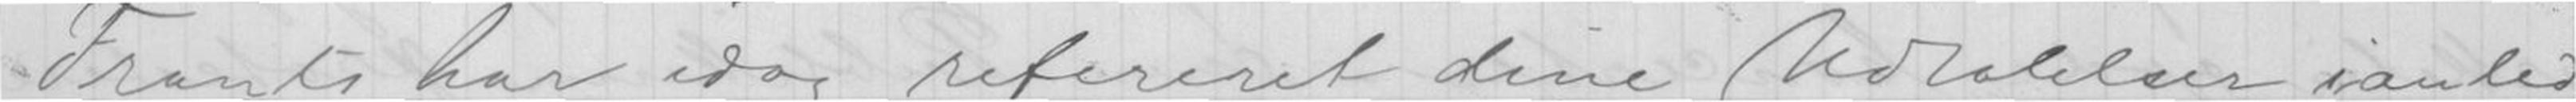Frants har idag refereret dine Udtalelser iandled-
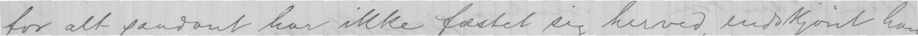for alt saadant, har ikke fæstet sig herved, endskjønt han
